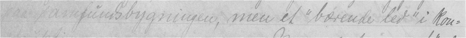paa samfundsbygningen, men et "bærende led" i kon-
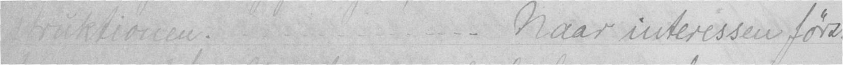struktionen. - - - - - - - - - - - - - - Naar interessen først
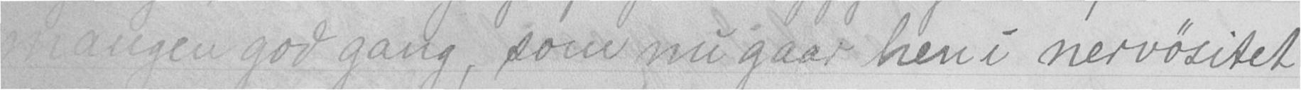mangen god gang, som nu gaar hen i nervøsitet
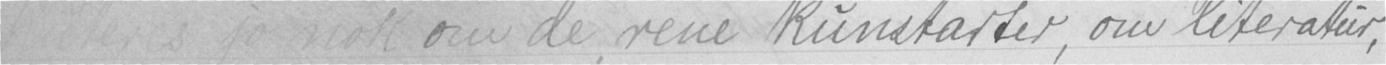kuteres jo nok om de rene kunstarter, om literatur,
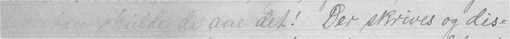hvordan skulde de ane det! Der skrives og dis-
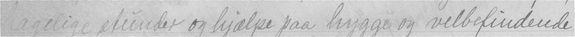hagelige stunder og hjælpe paa hygge og velbefindende
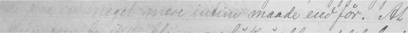de paa en meget mere intim maade end før. At
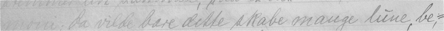moni, da vilde bare dette skabe mange lune, be-
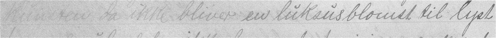kunsten da ikke bliver en luksus blomst til lyst
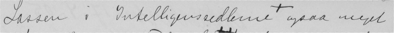Lassen i Intelligenssedlerne ogsaa meget
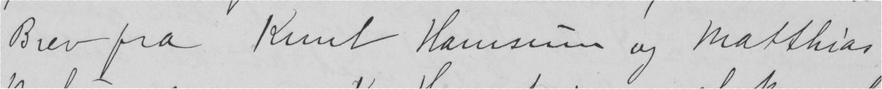Brev fra Knut Hamsum og Matthias
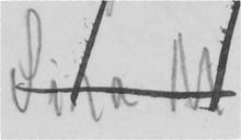Sina M.
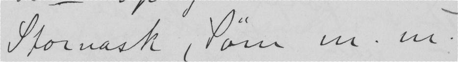Storvask, Søm m. m.
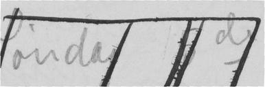Søndag 5de
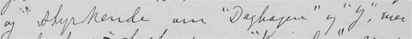og styrkende om "Dagbogen" og "J." mer
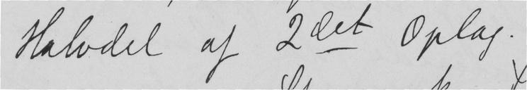Halvdel af 2det Oplag.
